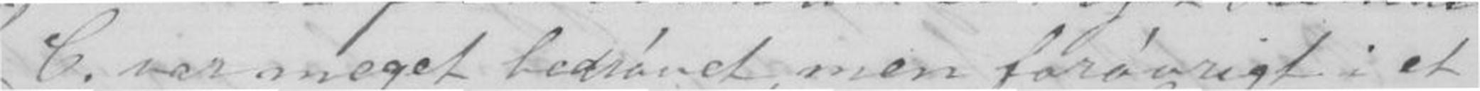C. var meget bedrøvet, men forøvrigt i et
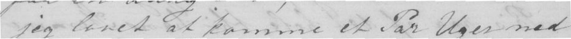jeg lovet at komme et Par Uger ned
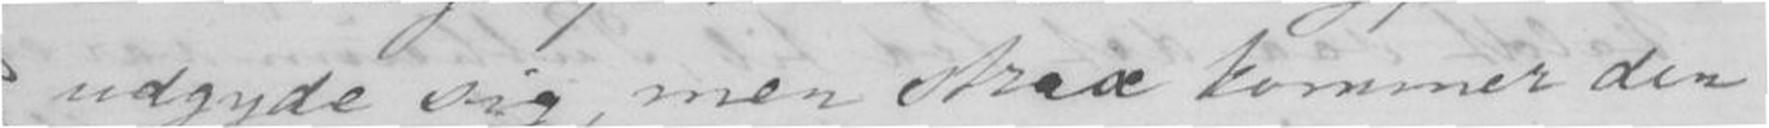udgyde sig, men strax kommer den
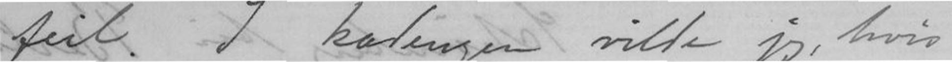feil. I kadengen vilde jeg, hvis
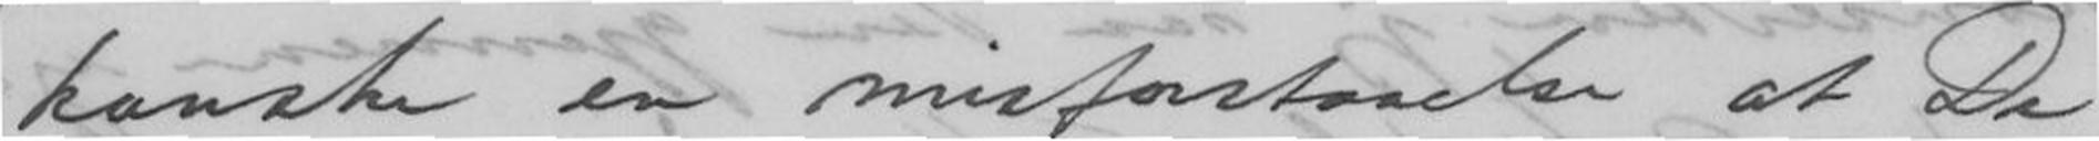kanske en misforstaaelse at De
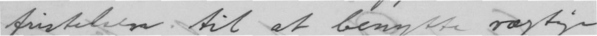fristelsen til at benytte vægtige
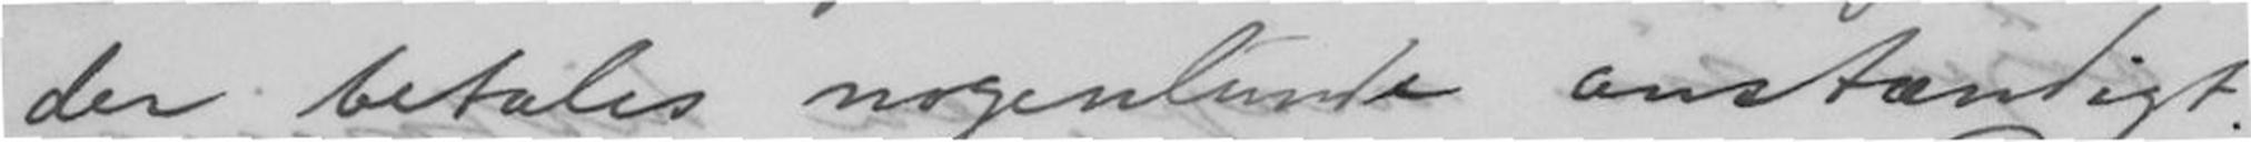at der betales nogenlunde anstændigt.
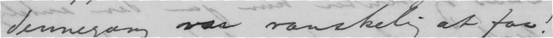dennegang var vanskelig at faa!
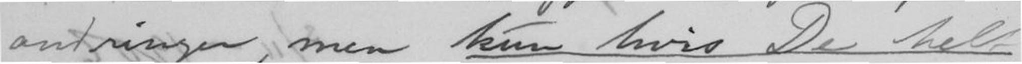andringer, men kun hvis De helt
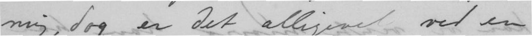mig, dog er det alligevel ved en
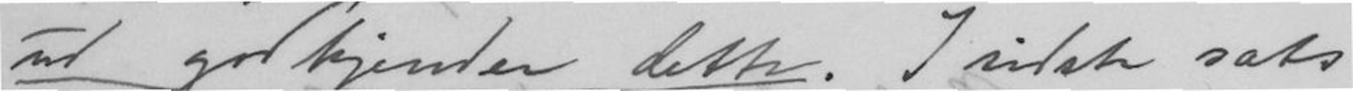ud godkjender dette. I sidste sats
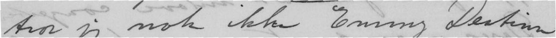tror jeg nok ikke Emmy Destinn
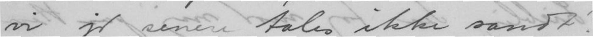vi jo senere tales, ikke sandt?
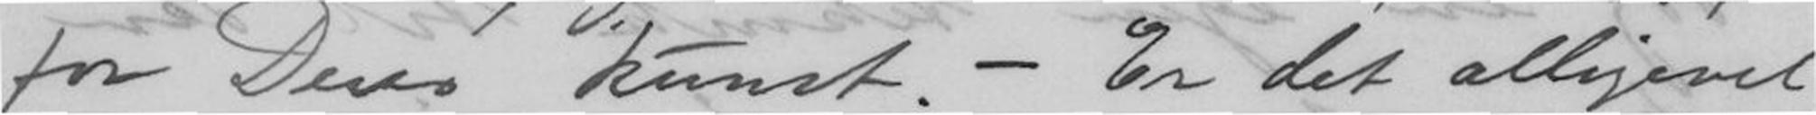for Deres kunst. - Er det alligevel
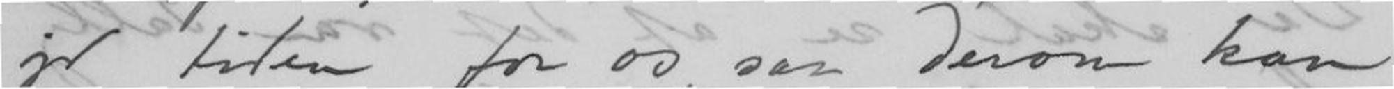jo tiden for os, saa derom kan
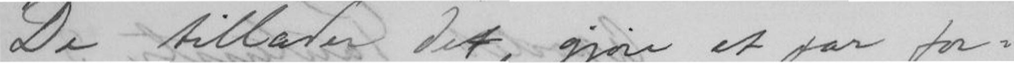De tillader det, gjøre et par for-
